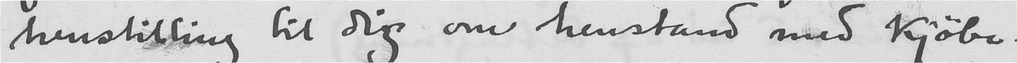henstilling til dig om henstand med kjøbe-
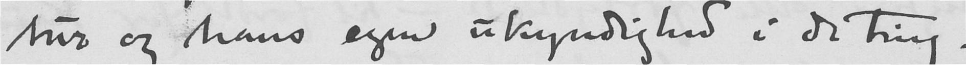tur og hans egen ukyndighed i de ting.
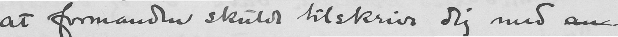at formanden skulde tilskrive dig med an-
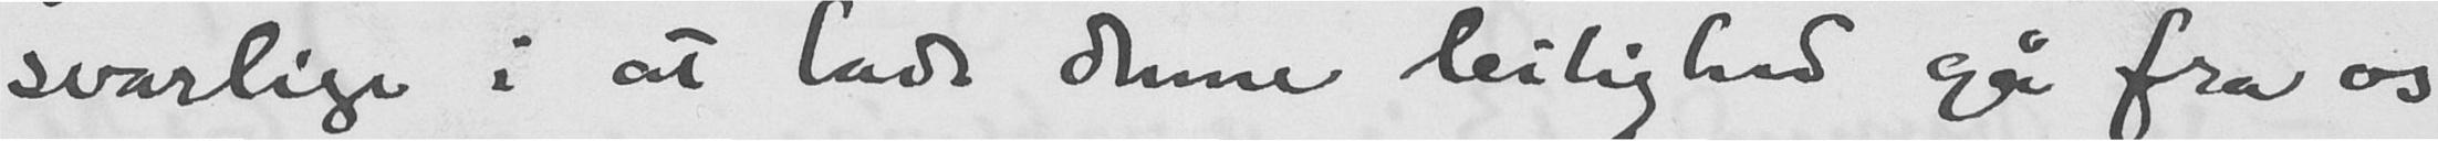svarlige i at lade denne leilighed gå fra os
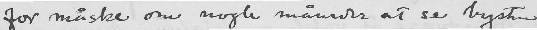for måske om nogle måneder at se bysten
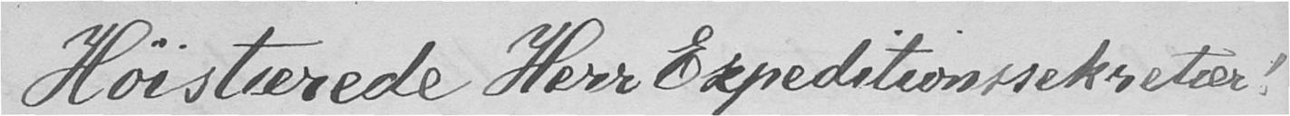Høistærede Herr Expeditionssekretær!
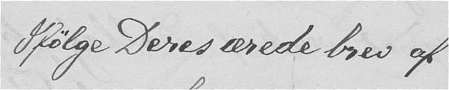Ifølge Deres ærede brev af
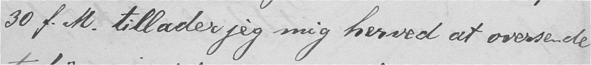30 f. M. tillader jeg mig herved at oversende
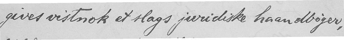gives vistnok et slags juridiske haandbøger,
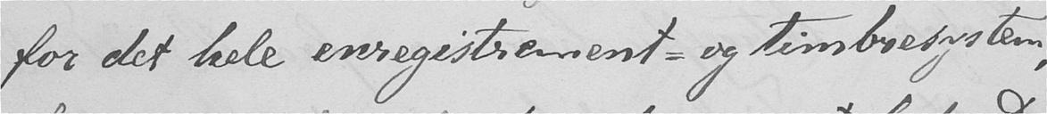for det hele enregistrement- og timbresystem,
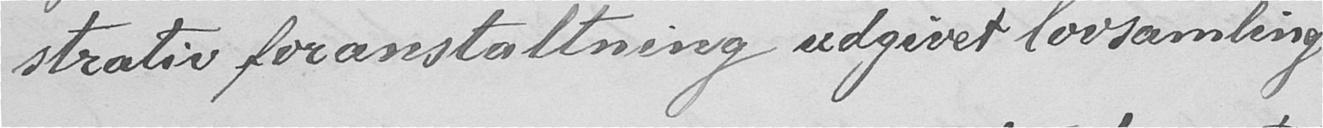strativ foranstaltning udgivet lovsamling
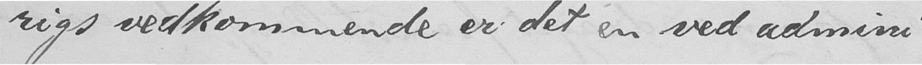rigs vedkommende er det en ved admini-
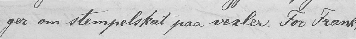ger om stempelskat paa vexler. For Frank-
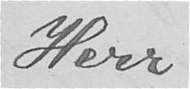Herr
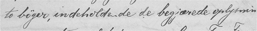to bøger, indeholdende de begjærede oplysnin-
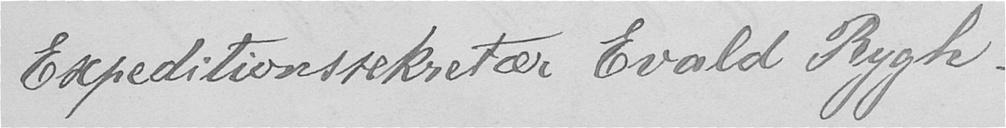Expeditionssekretær Evald Rygh.
